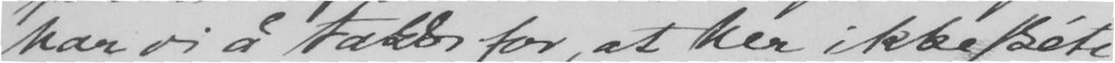har vi å takke for, at her ikke skéte
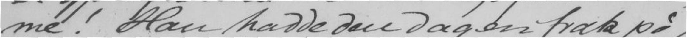me! Han hadde den dagen frak på,
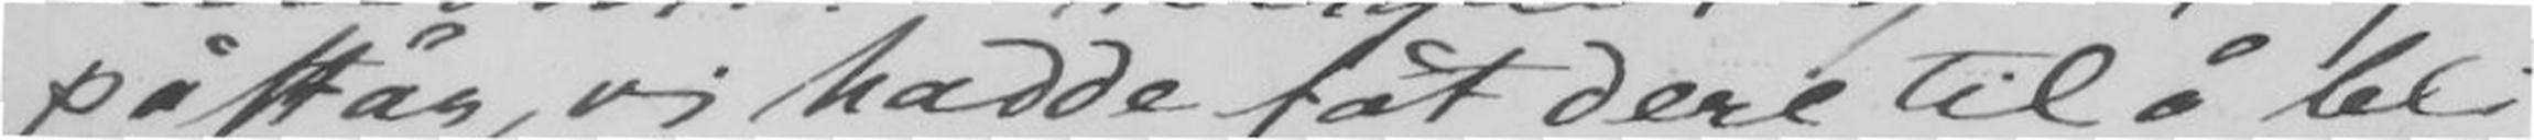påstår, vi hadde fåt dere til å bli
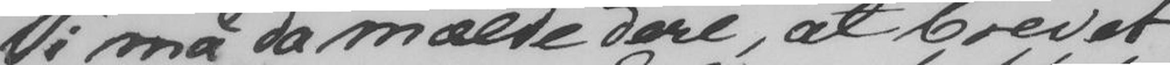Vi må da mælde dere, at brevet er
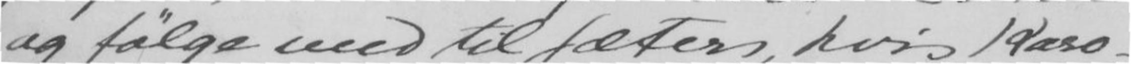og følge med til sæters, hvis Karo-
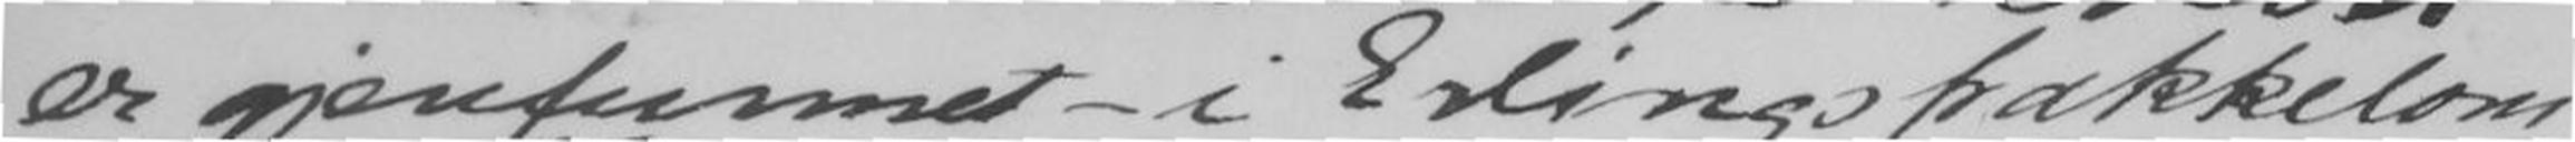gjenfunnet - i Erlings frakkelom-
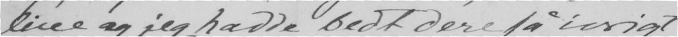line og jeg hadde bedt dere så ivrigt
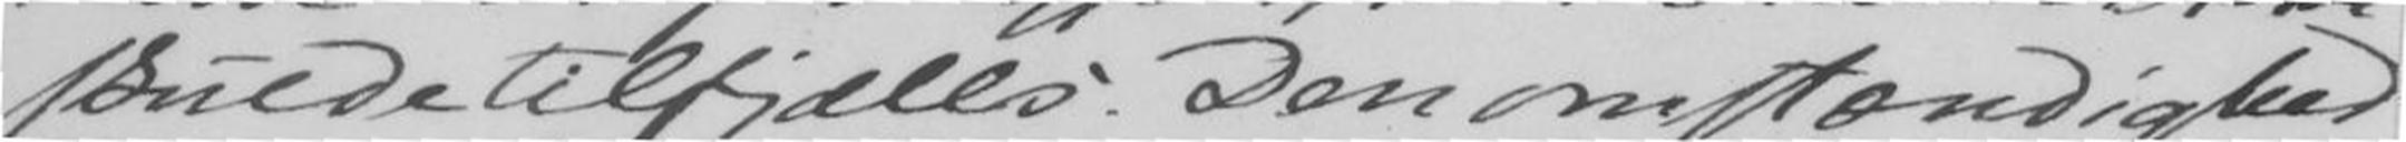skulde tilfjælls. Den omstændighed
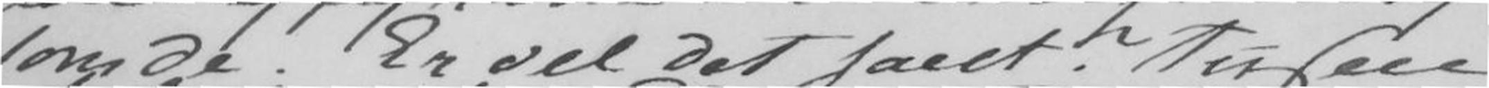som de. Er vel det sant? Tusen
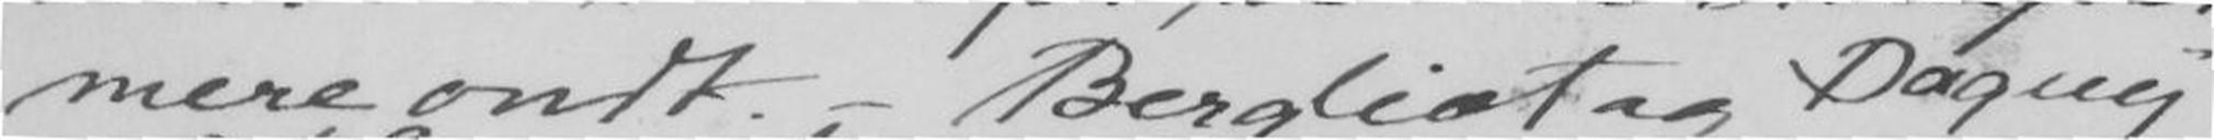mere ondt. - Bergliot og Dagny
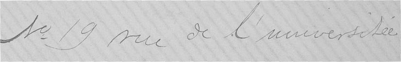No. 19 rue de l'universitée
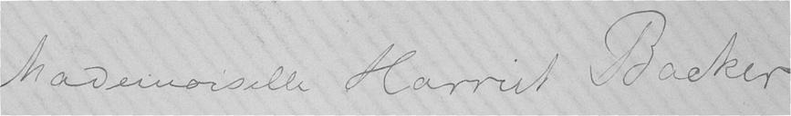Mademoiselle Harriet Backer
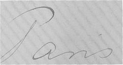Paris
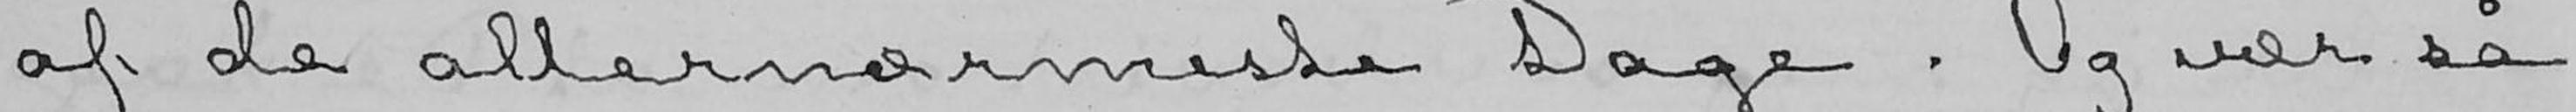af de allernærmeste Dage. Og vær så
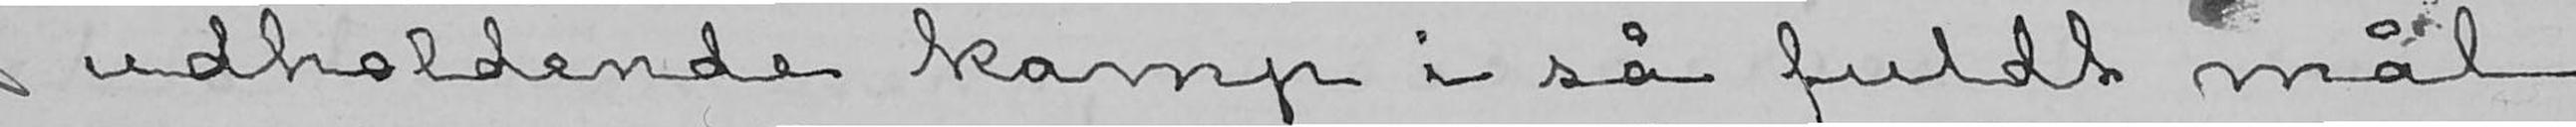udholdende kamp i så fuldt mål
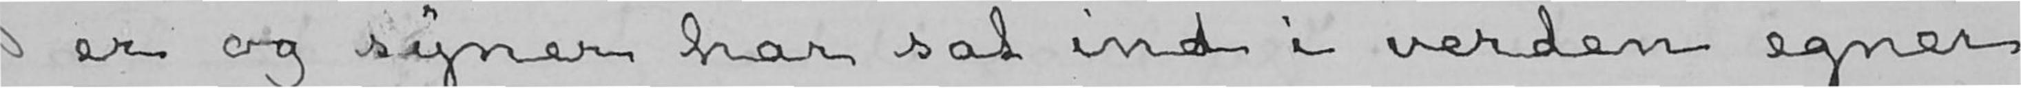er og syner har sat ind i verden egner
